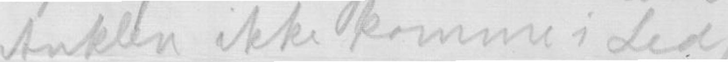Ankelen ikke komme i Led,
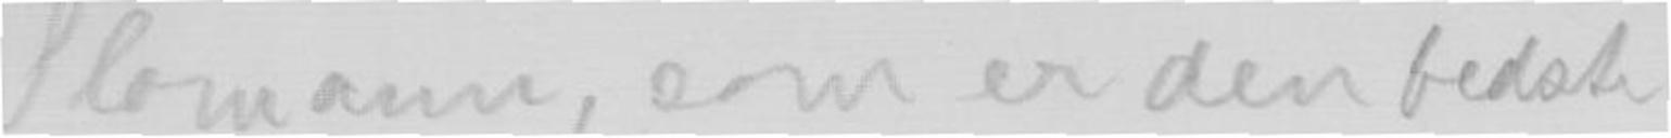Slomann som er den bedste
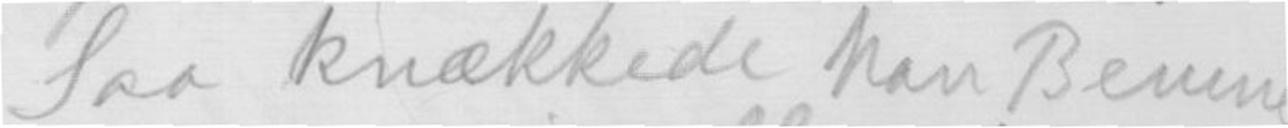Saa knækkede han Benene
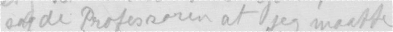sagde Professoren at jeg maatte
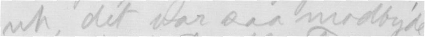uh, det var saa modbyde-
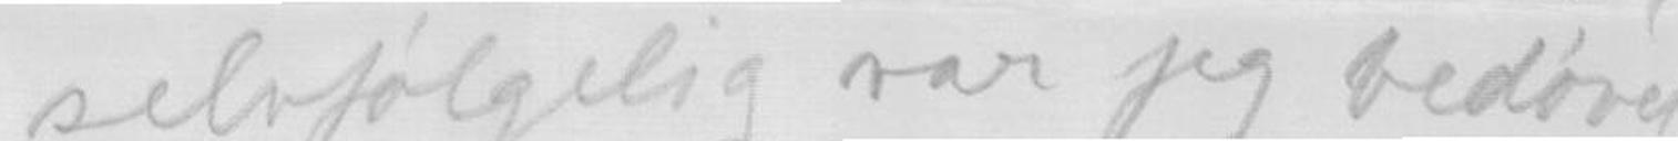selvfølgelig var jeg bedøvet
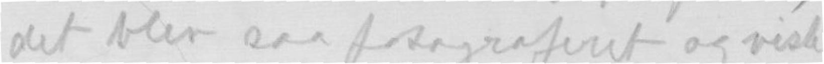det blir saa fotograferet og viste
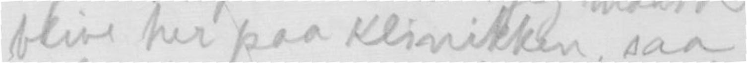blive her paa Klinikken, saa
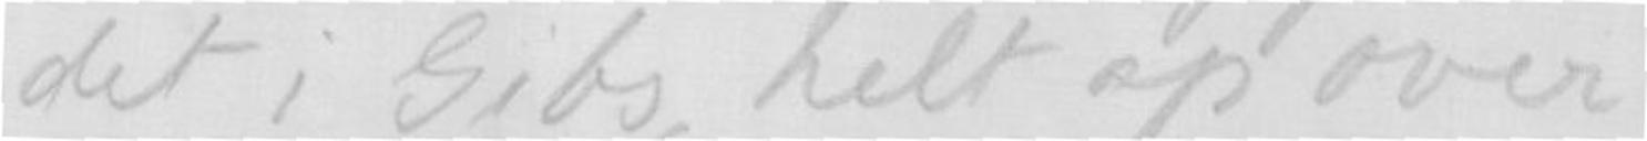det i Gibs, helt op over
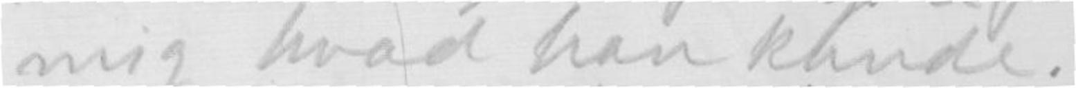mig hvad han kunde.
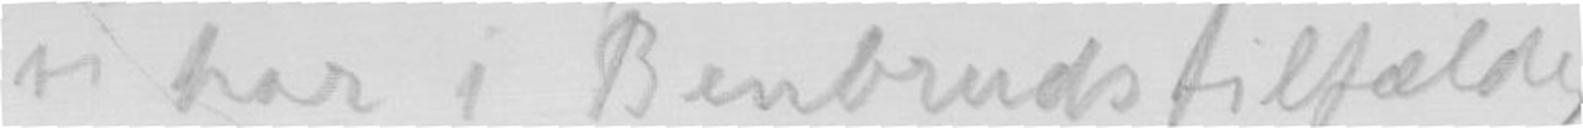vi har i benbrudstilfælde,
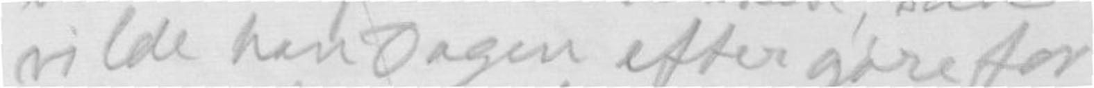vilde han Dagen efter gøre for
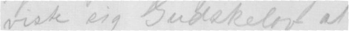viste sig Gudskelov at
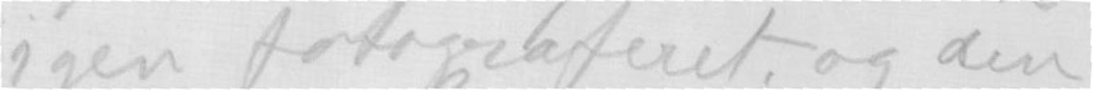igen fotograferet, og den
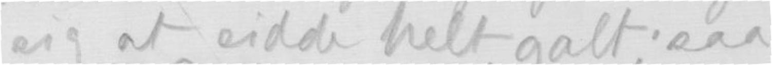sig at sidde helt galt; saa
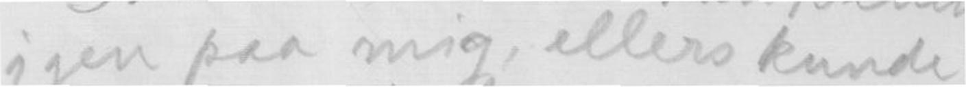igen paa mig, ellers kunde
